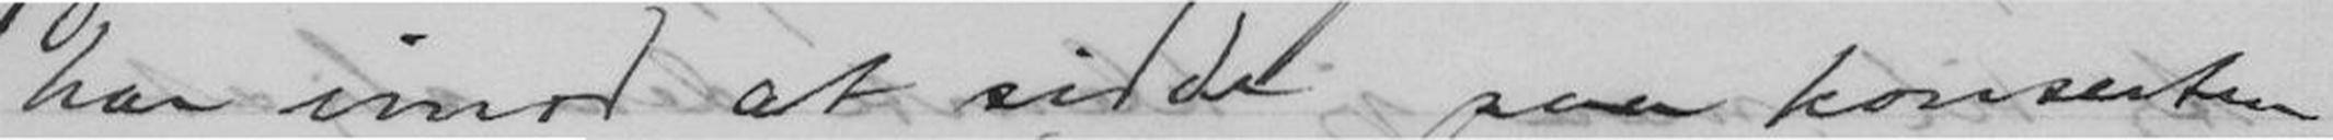har imod at sidde paa konserten
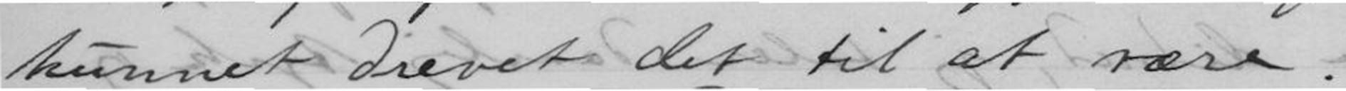kunnet drevet det til at være. -
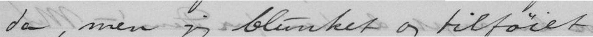da, men jeg blunket og tilföiet
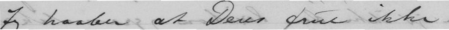Jeg haaber at Deres frue ikke
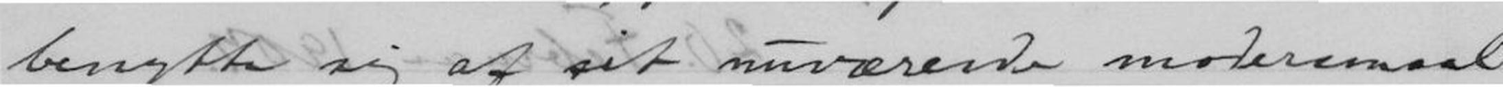benytte sig af sit nuværende modersmaal:
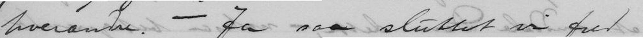hverandre. - Ja saa sluttet vi fred
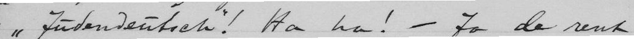"Judendeutsch"! Ha ha! - Jo de rent
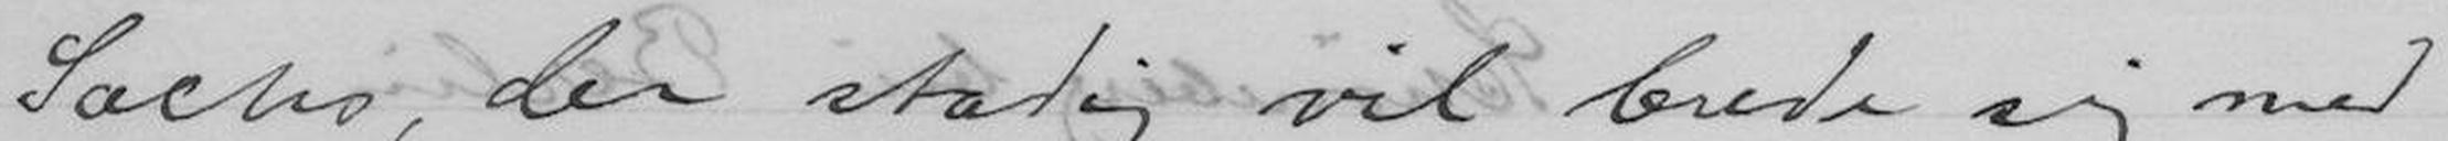Sachs, der stadig vil brede sig med
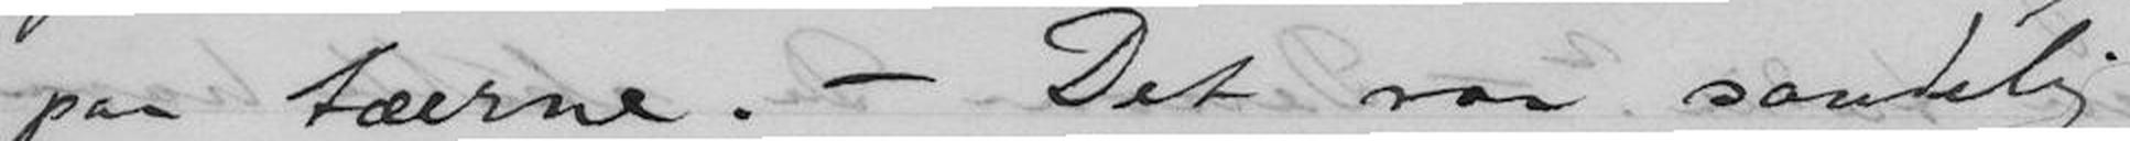paa tæerne. - Det var sandelig
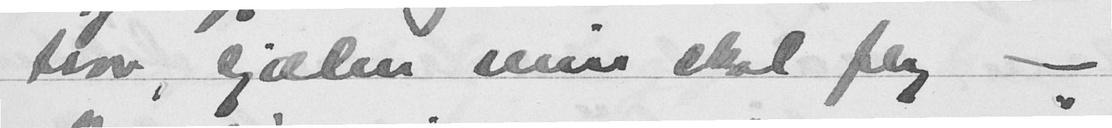tror, sjælen min skal fly -
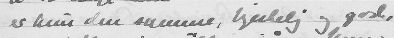er hun den samme, hjertelig og god,
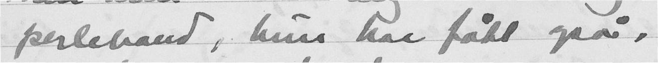perleband, hun har fått også,
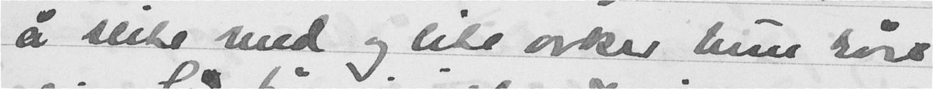å slite med og lite orker hun røre
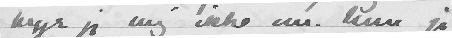bryr jeg mig ikke om. Men jeg
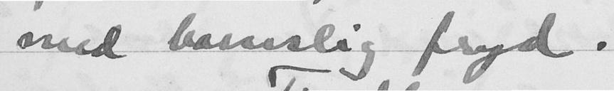med barnslig fryd.
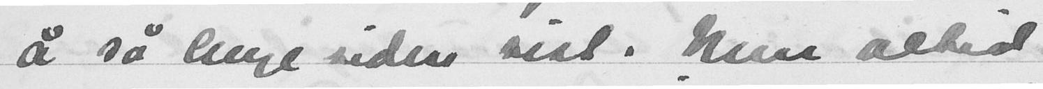Å så lenge siden sist. Men altid
